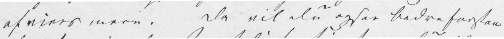afrives mere. Da vil Du ogsaa bedre forstaa
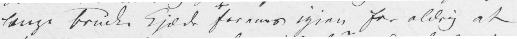lange brudte Kjæde forenes igjen for aldrig at
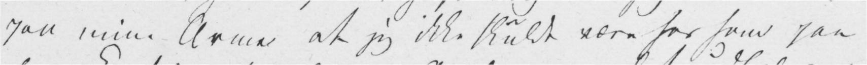paa mine Arme at jeg ikke skulde være hos ham paa
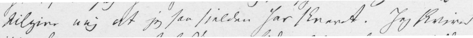tilgive mig at jeg saa sjelden har skrevet. Jeg skriver
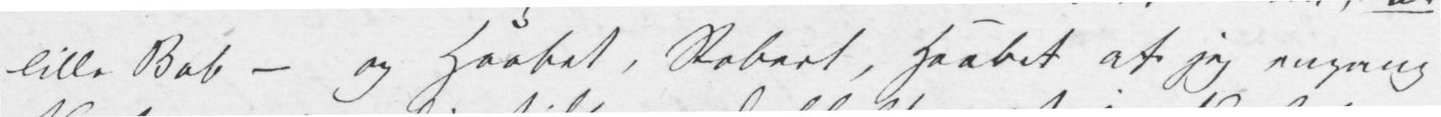lille Bob – og Haabet, Robert, Haabet at jeg engang
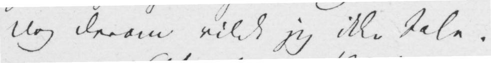Dog derom vilde jeg ikke tale.
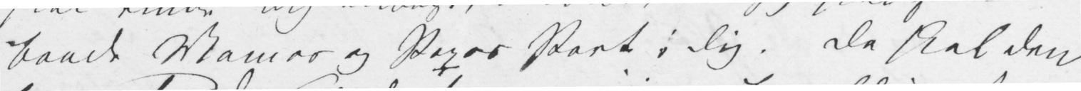baade Mamas og Papas Part i Dig. Da skal den
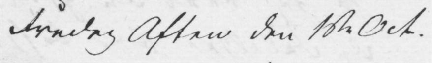Fredag Aften den 1ste Oct.
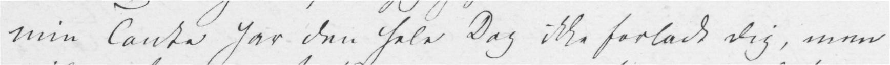min Tanke har den hele Dag ikke forladt Dig, men
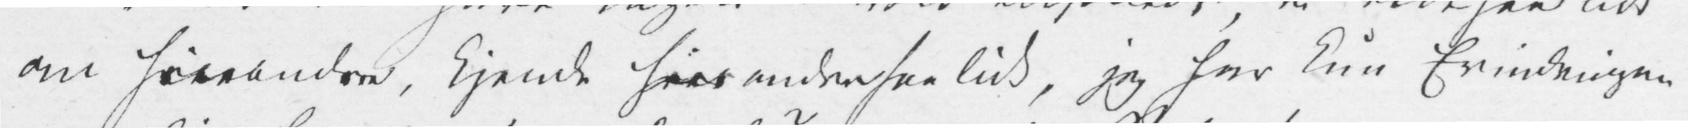om hinanden, kjende hinanden saa lidt, jeg har kun Erindringen
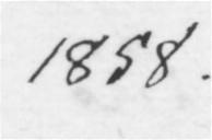1858.
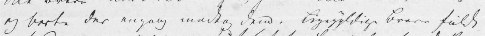og borte der engang modtog dem. Ligegyldige Breve fulde
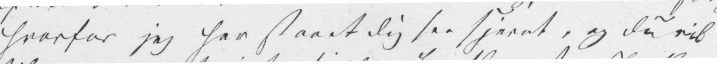hvorfor jeg har staaet Dig saa fjernt, og Du vil
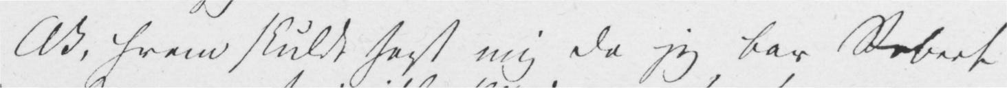Ak, hvem skulde sagt mig da jeg bar Robert
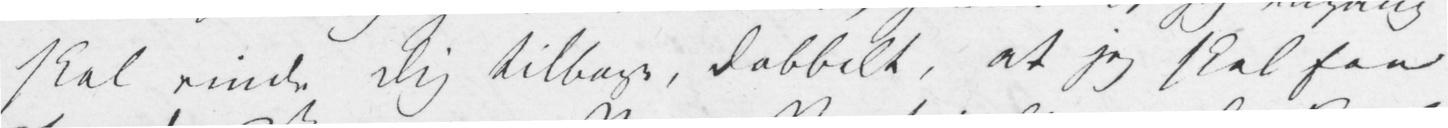skal vinde Dig tilbage, dobbelt, at jeg skal faa
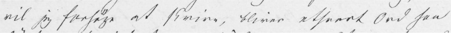vil jeg forsøge at skrive, bliver ethvert Ord saa
